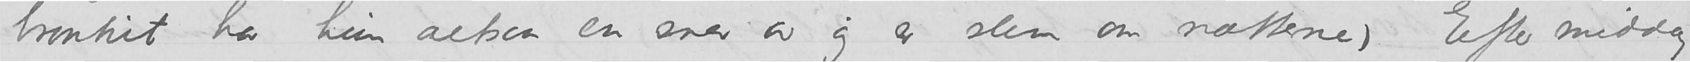bronkit har hun altsaa en snæev av og er slem om nætterne) Efter middag
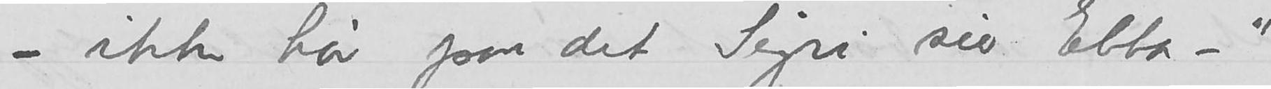– ikke hør paa det Sigri sier Ebba –«.
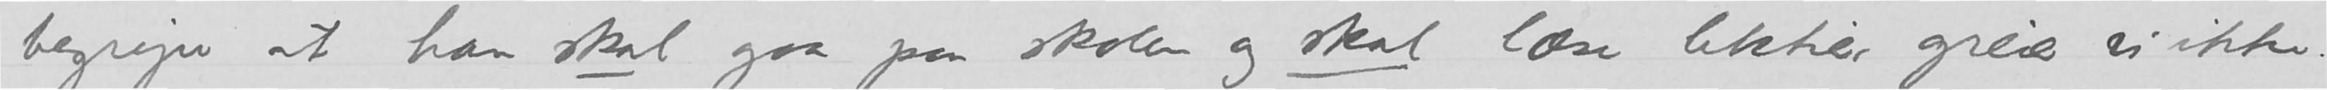begripe at han skal gaa paa skolen og skal læse lektier greier vi ikke.
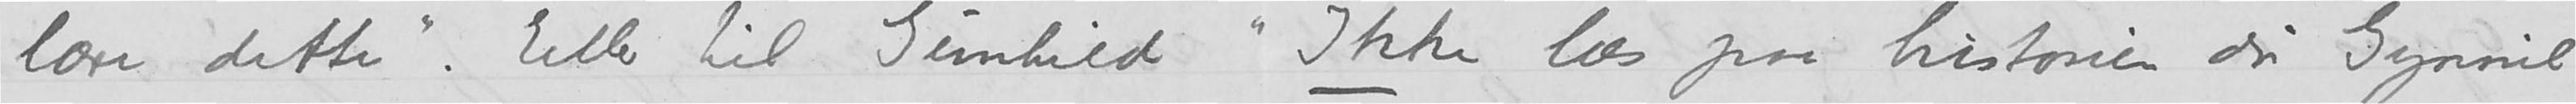lære dette». Eller til Gunhild «Ikke læs paa historien du Gynnil
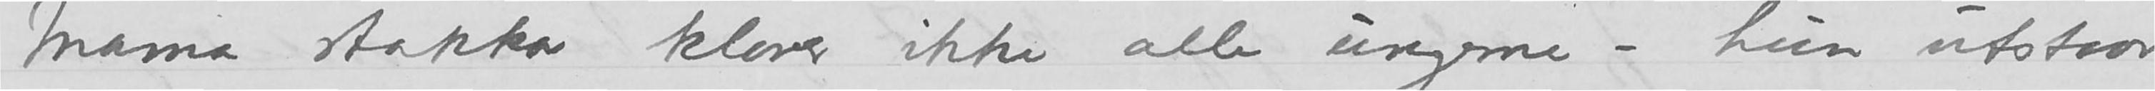Mama stakkar klarer ikke alle ungerne – hun utstaar
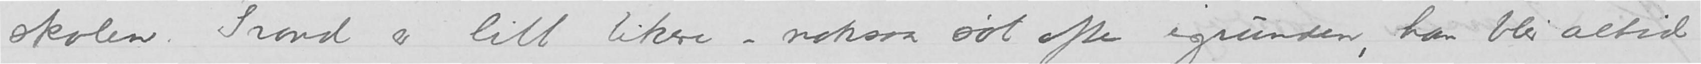skolen. Trond er litt likere – noksaa søt ofte igrunden, han blir altid
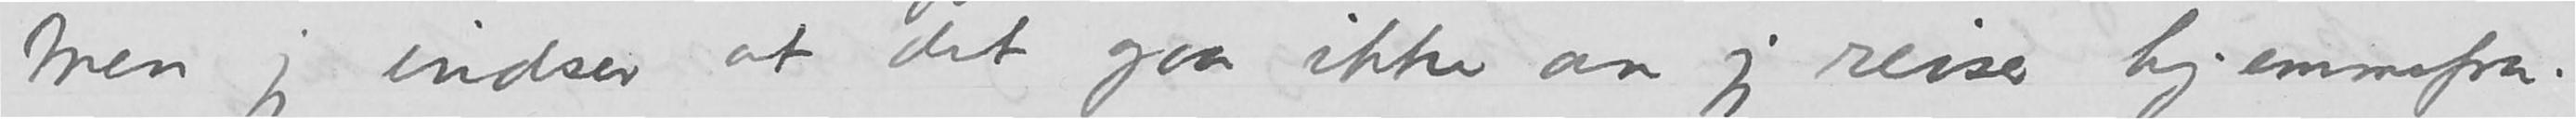Men jeg indser at det gaar ikke an jeg reiser hjemmefra.
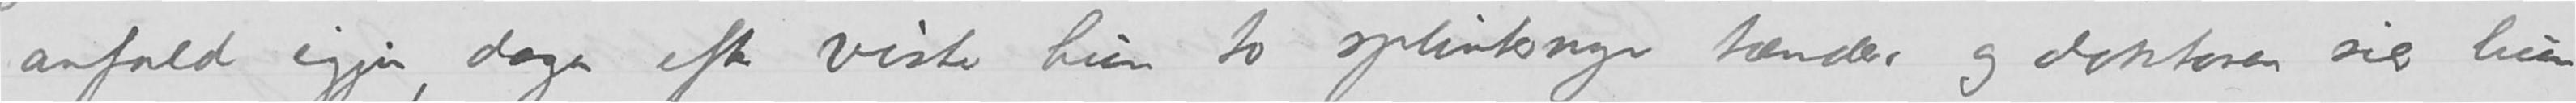anfald igjen, dagen efter viste hun to splinternye tænder, og doktoren sier hun
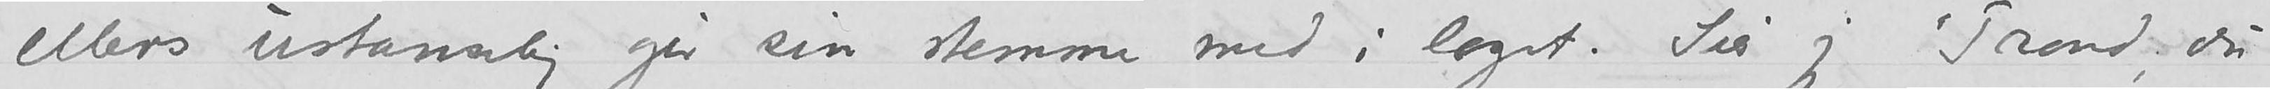ellers ustanselig gir sin stemme med i laget. Sier jeg «Trond, du
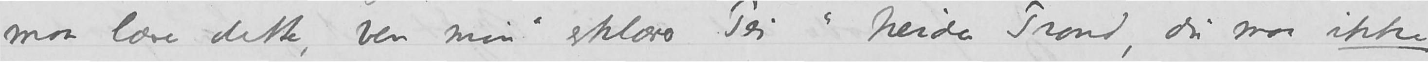maa lære dette, ven min» erklærer Pei «Neida Trond, du maa ikke
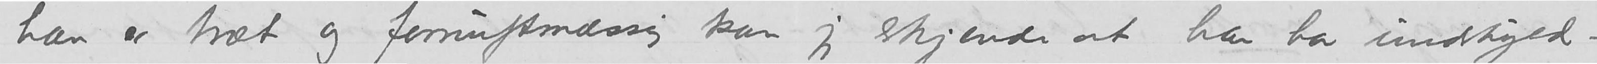han er træt og fornuftsmæssig kan jeg erkjende at han har undskyld-
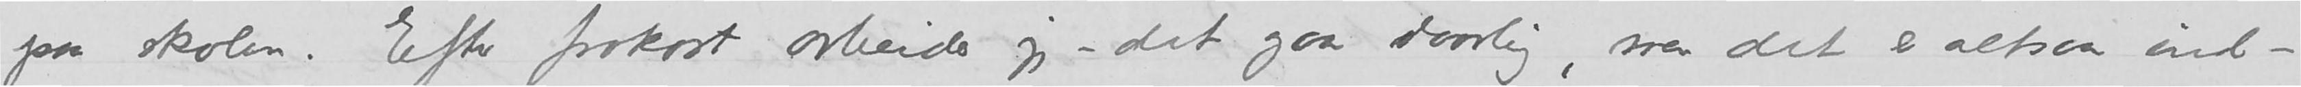paa skolen. Efter frokost arbeider jeg – det gaar daarlig, men det er altsaa ind-
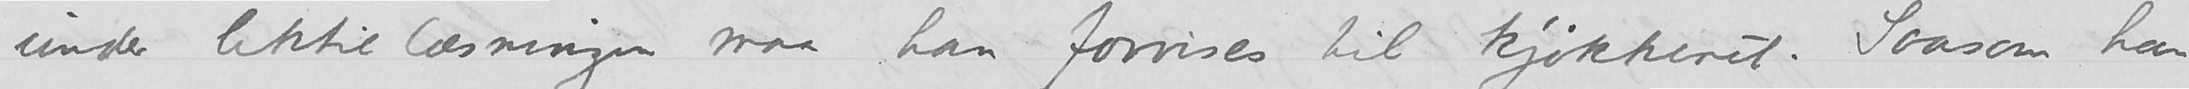under lektielæsningen maa han forvises til kjøkkenet. Saasom han
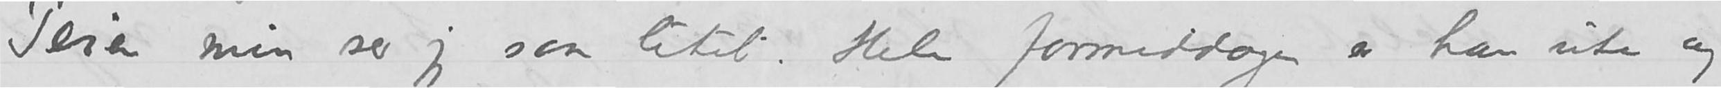Peien min ser jeg saa litet. Hele formiddagen er han ute og
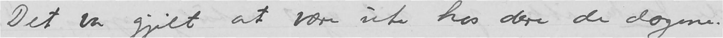Det var gjilt at være ute hos dere de dagene.
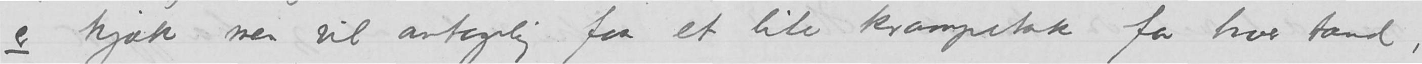er kjæk men vil antagelig faa et lite krampetak for hver tand,
